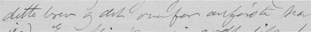dette brev og det overfor anførte har
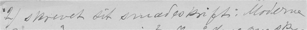2) skrevet sit smædeskrift: Moderne
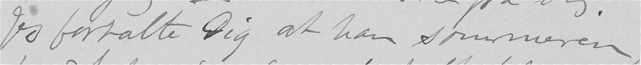Jeg fortalte Dig at han sommeren
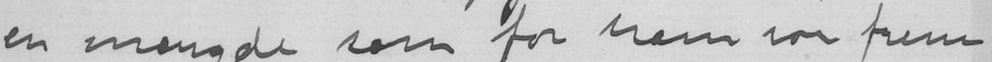en mængde, som for ham var frem
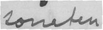sonaten
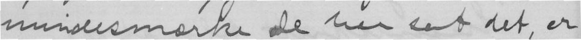mindesmærke De har sat det, er
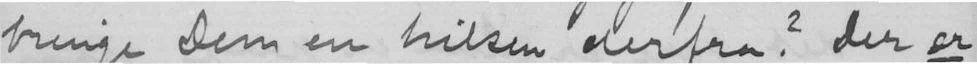bringe Dem en hilsen derfra? Der er
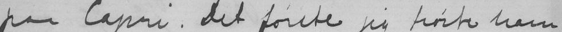paa Capri. Det første jeg hørte ham
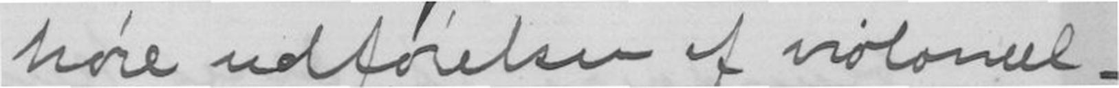høre udførelsen af violoncel-
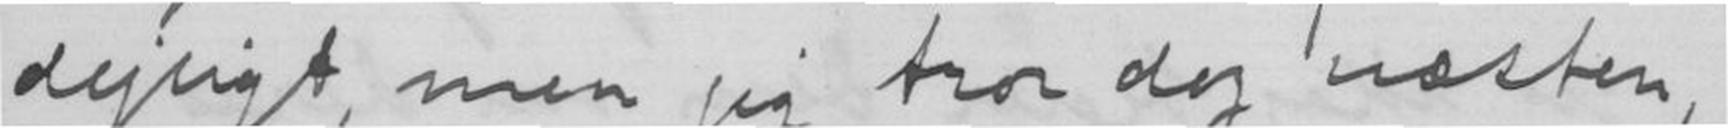dejligt, men jeg tror dog næsten,
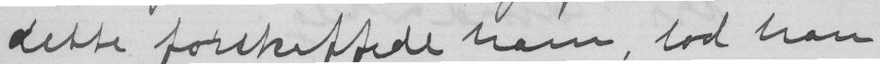dette forskaffede ham, lod han
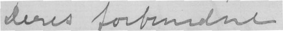Deres forbundne
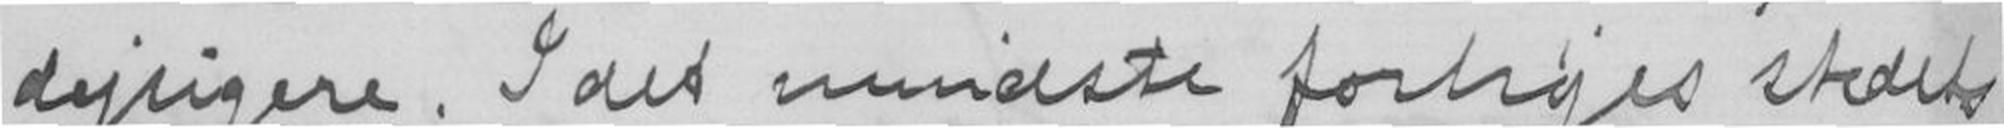dejligere. I det mindste forhøjes stedets
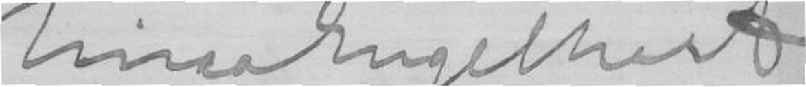Nina Engelhart.
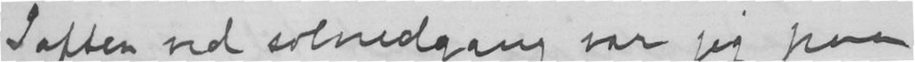I aften ved solnedgang var jeg paa
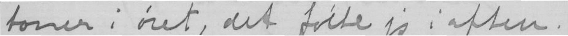toner i øret, det følte jeg i aften.
